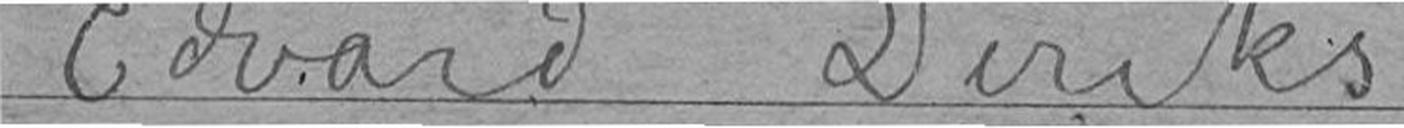Edvard Diriks
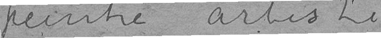peintre artiste
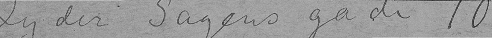Lyder Sagens gade 10
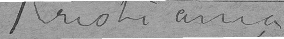Kristiania
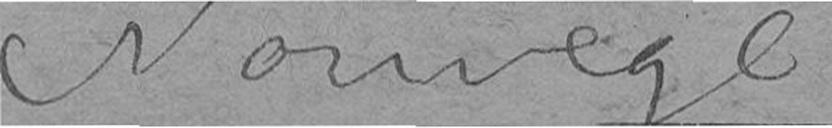Norwege
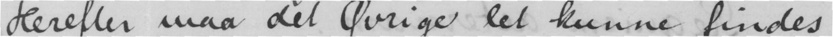Herefter maa det Øvrige let kunne findes,
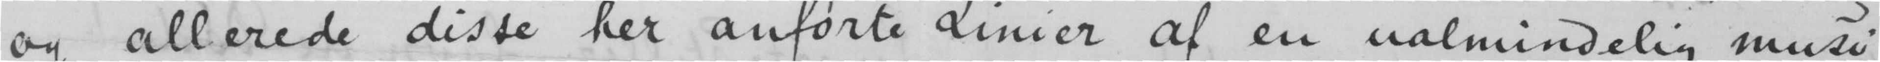og allerede disse her anførte Linier af en ualmindelig musi-
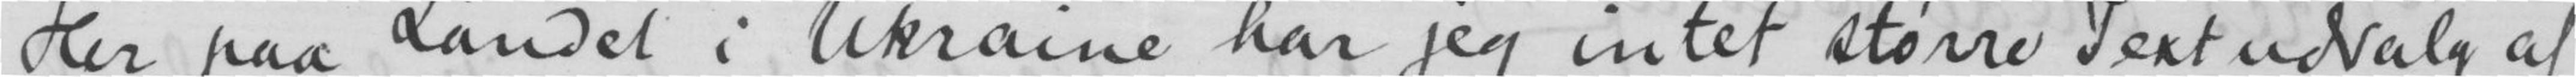Her paa Landet i Ukraine har jeg intet større Textudvalg af
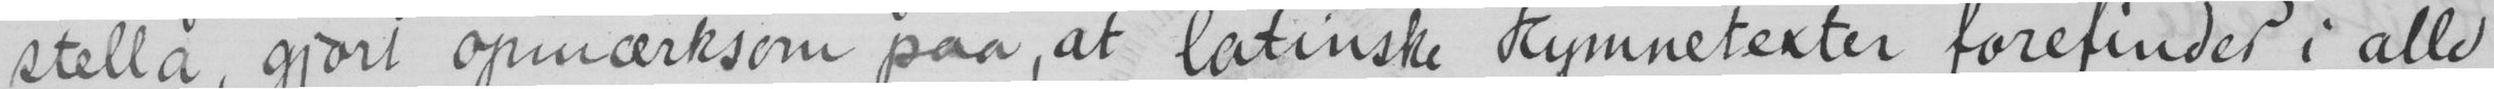stella, gjort opmærksom paa, at latinske Hymnetexter forefinder i alle
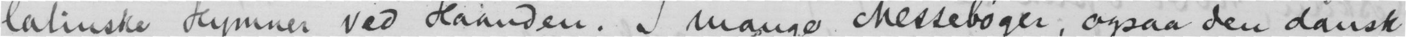latinske Hymner ved Haanden. I mange Messebøger, ogsaa den dansk
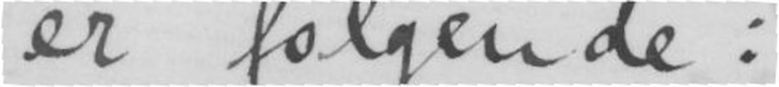er følgende:
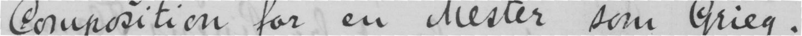Composition for en Mester som Grieg.
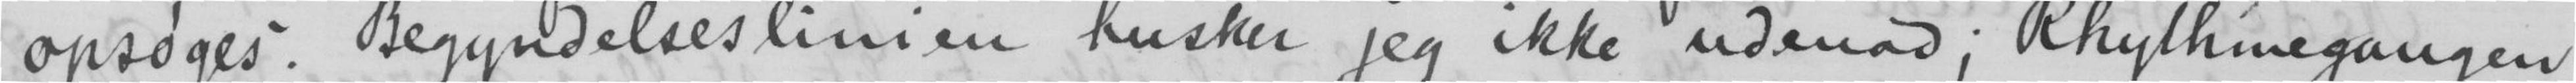Begyndelseslinien husker jeg ikke udenad; Rhytmegangen
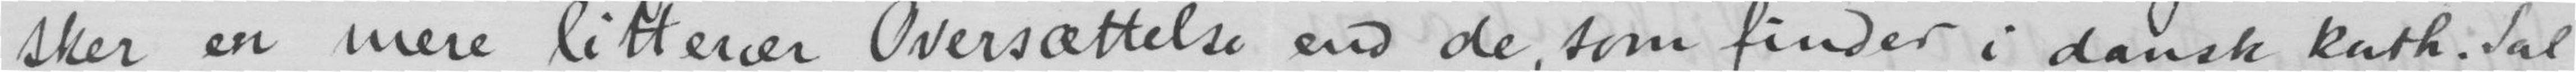sker en mere litterær Oversættelse end de, som finder i dansk kath Sal-
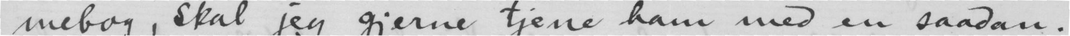mebog, skal jeg gjerne tjene ham med en saadan.
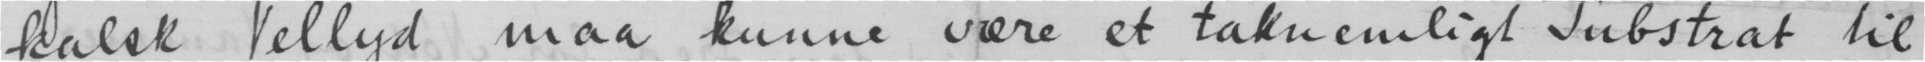kalsk Vellyd maa kunne være et taknemligt Substrat til
